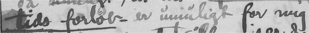tids forløb - er umuligt for mig
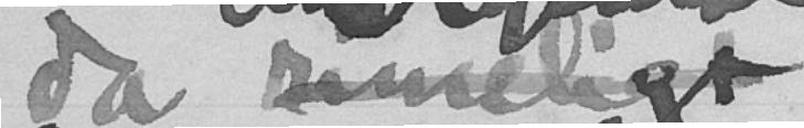da
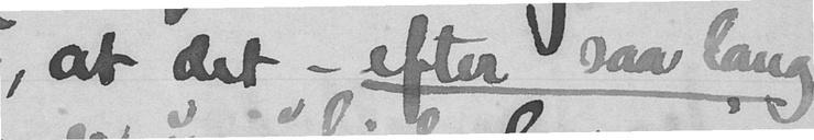, at det - efter saa lang
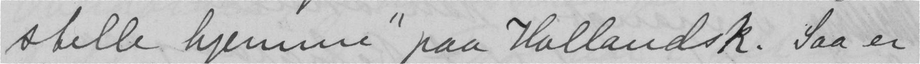stelle hjemme" paa Hollandsk. Saa er
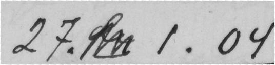27.  1.04
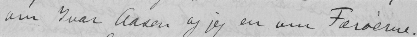om Ivar Aasen og jeg en om Færøerne.
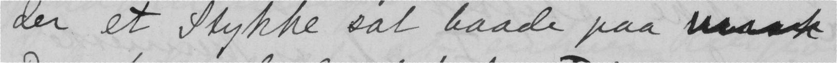der et Stykke sat baade paa
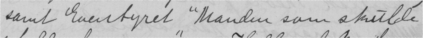samt Evenrtyret "Manden som skulde
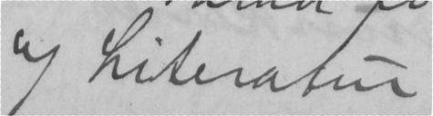og Literatur
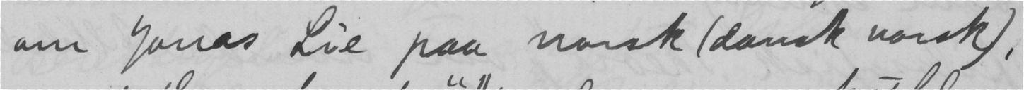om Jonas Lie paa norsk (dansk norsk),
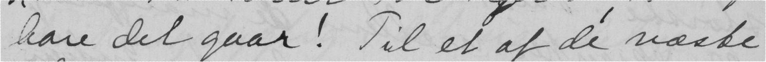bare det gaar! Til et af de næste
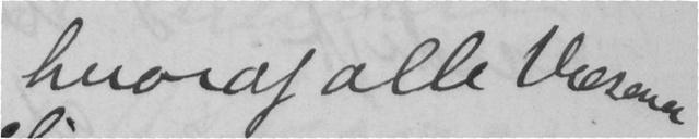hvoraf alle væsener
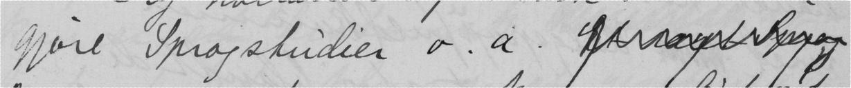gjøre Sprogstudier o. a.
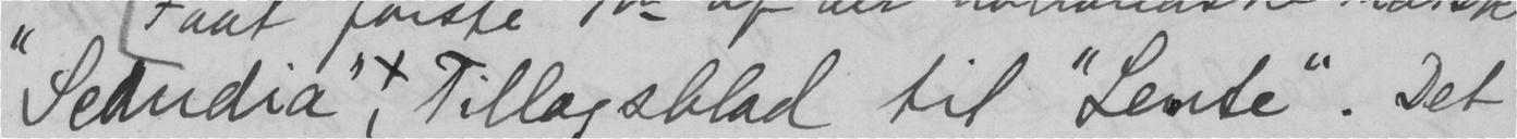"Scandia", Tillægsblad til "Leute". Det
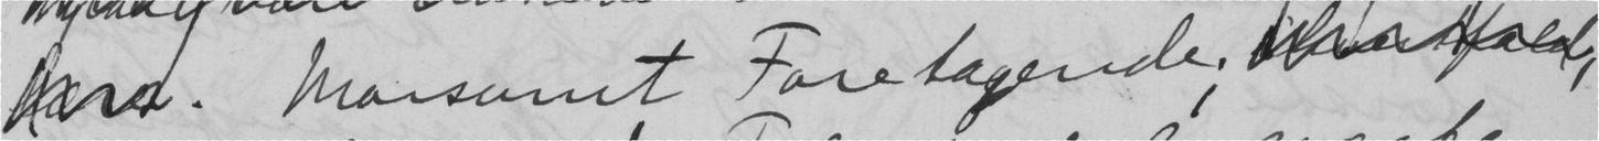. Morsomt Foretagende ,
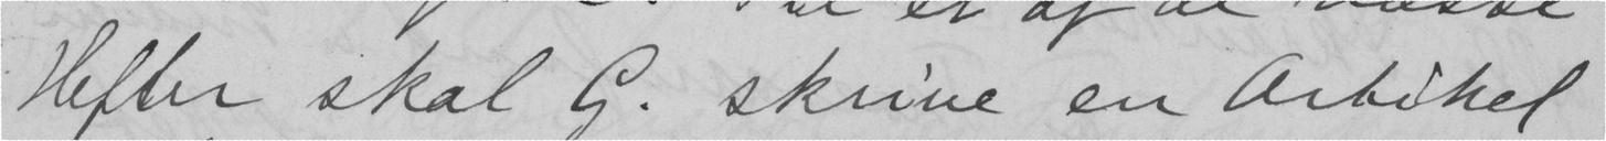Hefter skal G. skrive en Artikel
